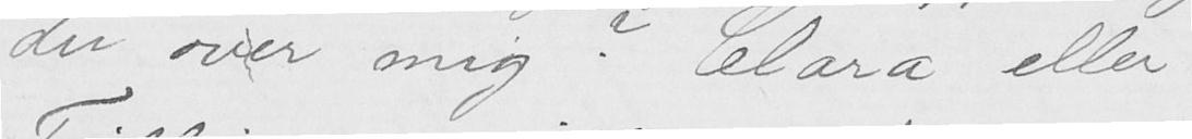du over mig? Clara eller
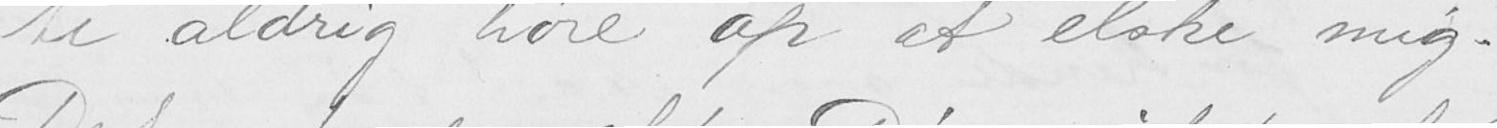te aldrig høre op at elske mig.
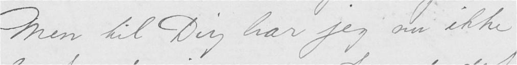Men til Dig har jeg nu ikke
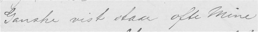Ganske vist staar ofte mine
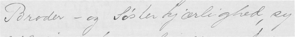Broder- og Søsterkjærlighed, sy-
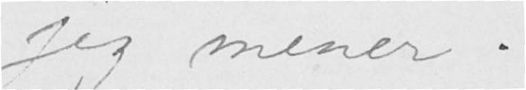jeg mener.
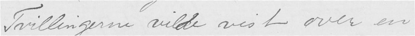Tvillingerne vilde vist over en
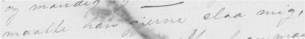maatte han gjerne slaa mig,
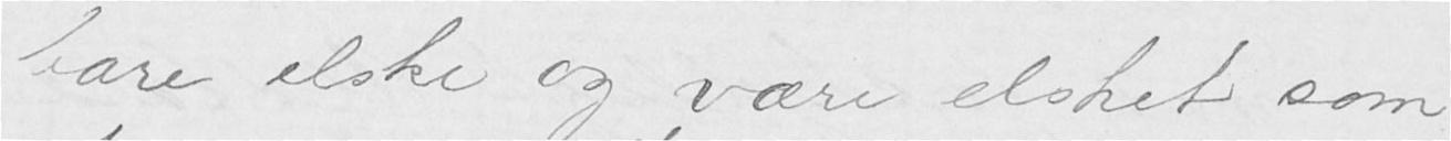bare elske og være elsket som
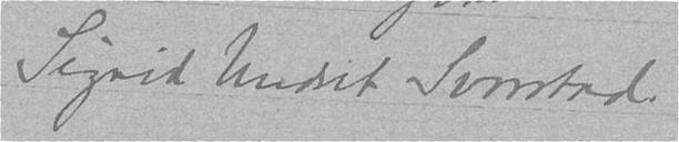Sigrid Undset Svarstad.
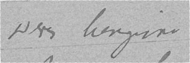Deres hengivne
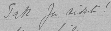Tak for sidst!
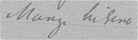Mange hilsener
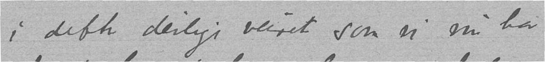i dette deilige veiret, som vi nu har
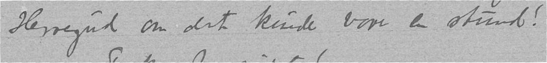Herregud om det kunde vare en stund!
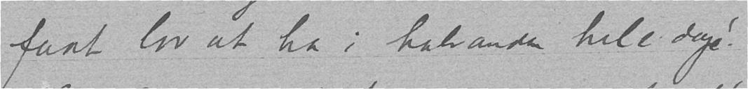faat lov at ha i halvanden hele dage!
